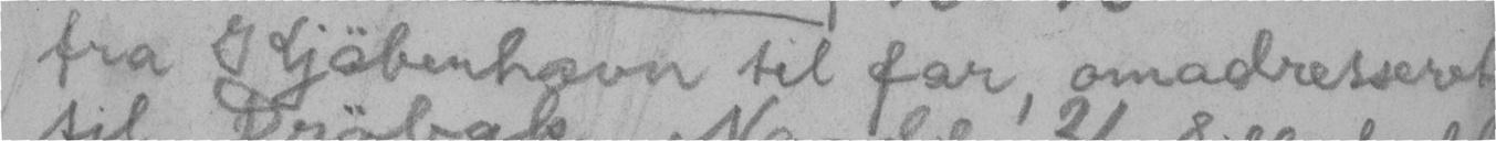fra Kjøbenhavn til far, omadræsseret
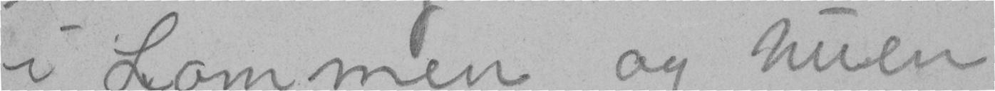i Lommen og huen
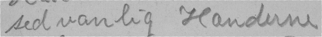sed vanlig Hænderne
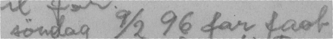søndag 9/2 96 far faet
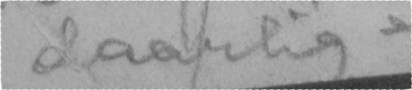daarlig
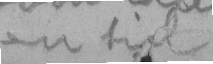er trist
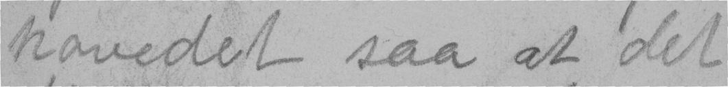hovedet saa at det
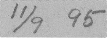11/9 95
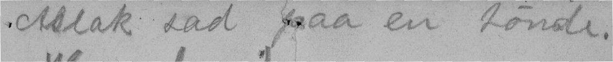Aslak sad paa en tønde.
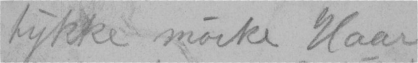tykke mørke Haar
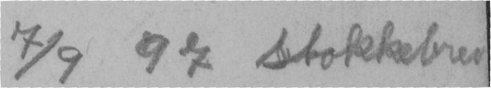7/9 97 Stokkebrev
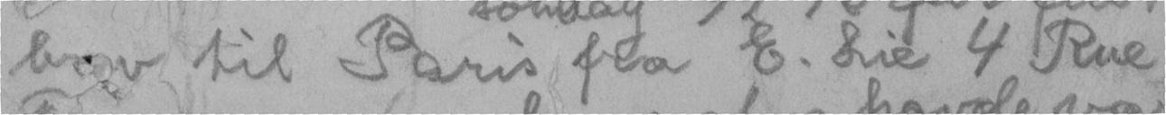brev til Paris fra E. vie 4 Rue
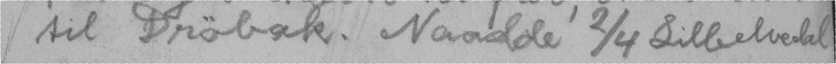til Drøbak. Naadde 2/4 Lilleelvedal
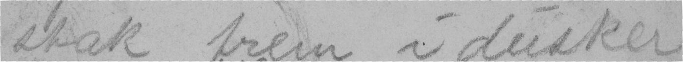stak frem i dusker
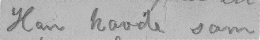Han havde som
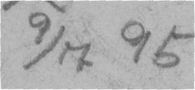9/17 95
